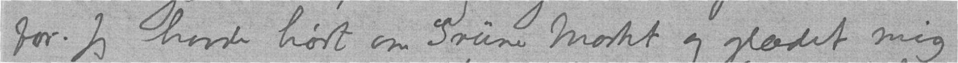for. Jeg havde hørt om Grüne Markt og glædet mig
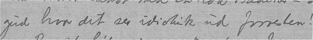gud hvor det ser idiotisk ud forresten!
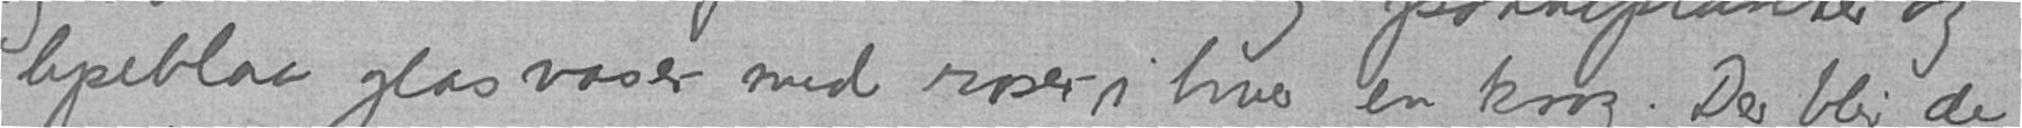lyseblaa glasvaser med roser - i hver en krog. Der blir de
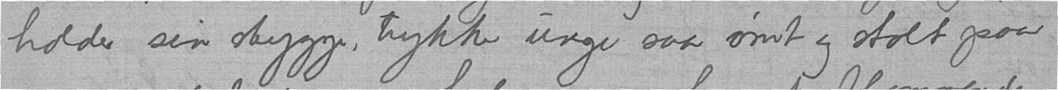holder sin stygge, tykke unge saa ømt og stolt paa
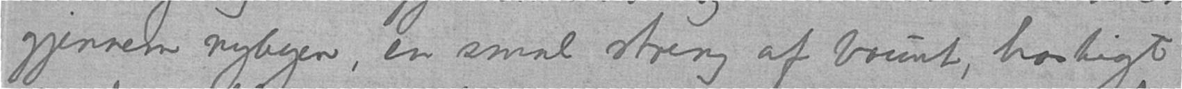gjennem nybyen, en smal streng af brunt, hastigt
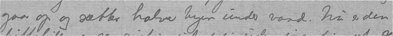gaar op og sætter halve byen under vand. Nu er den
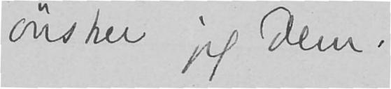ønsker jeg Dem.
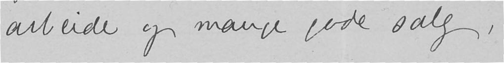arbeide og mange gode salg,
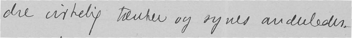dre virkelig tænker og synes anderledes
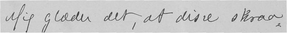Mig glæder det, at disse skraa-
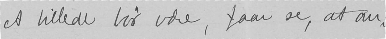et billede bør være, faar se, at an-
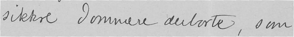sikkre dommere derborte, som
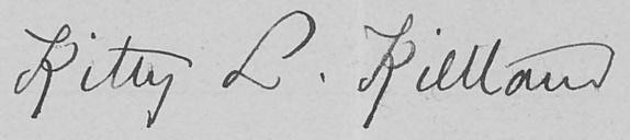Kitty L. Kielland.
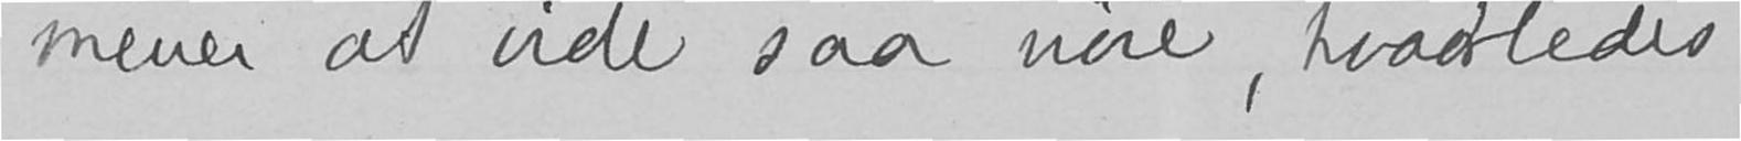mener at vide saa nøie, hvorledes
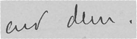end dem.
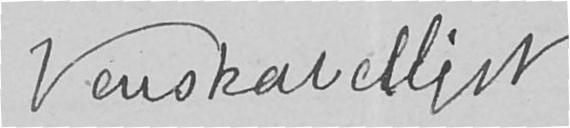Venskabelligst
